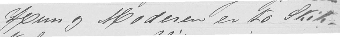Hun og Moderen er to Skik-
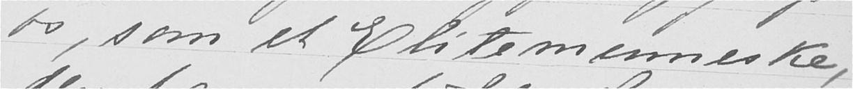os, som et Elitemeneske,
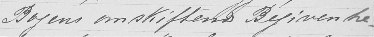Bogens omskiftende Begivenhe-
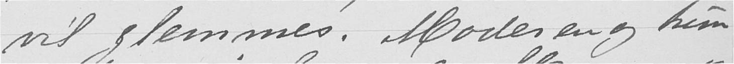vil glemmes. Moderen og hun
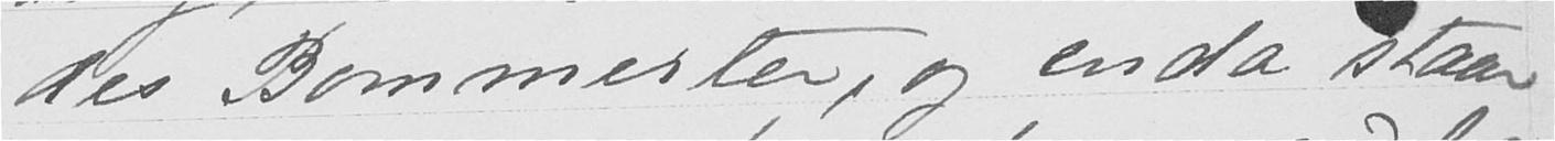des Bommerter, og enda staar
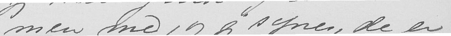men med, og jeg synes, de er
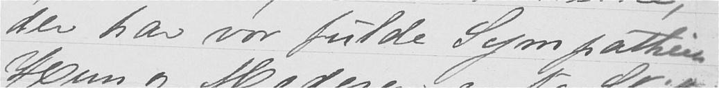der har vor fulde Sympathie.
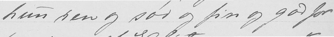hun ren og sød og fin og god for
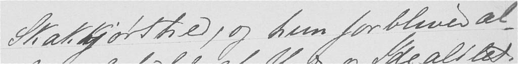Skakkjørthed, og hun forbliver al-
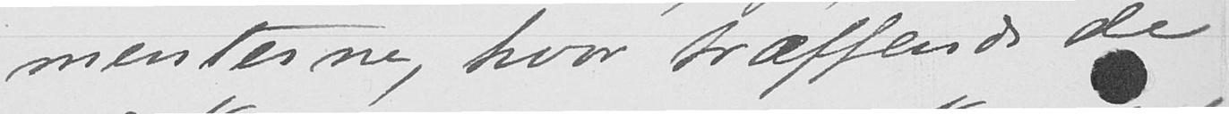menterne, hvor træffende de
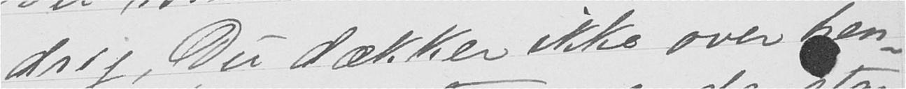drig, Du dækker ikke over hen-
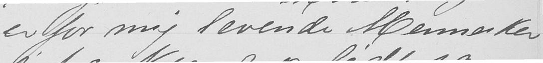er for mig levende Mennesker
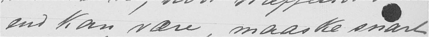end kan være, maaske snart
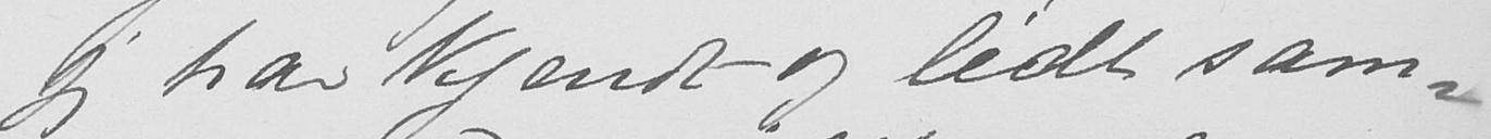jeg har kjendt og lidt sam-
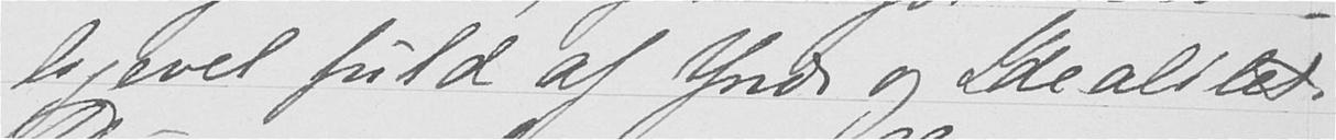ligevel fuld af ynde og Idealitet.
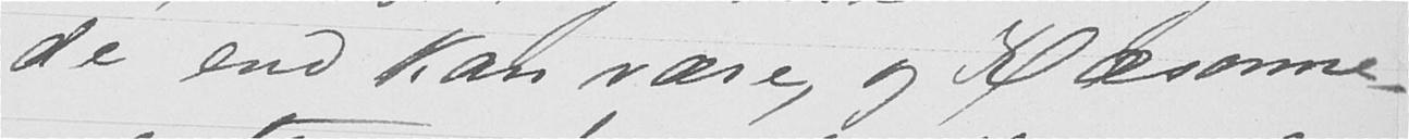de end kan være, og Ræsonne-
